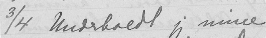3/4 Underholdt jeg mine
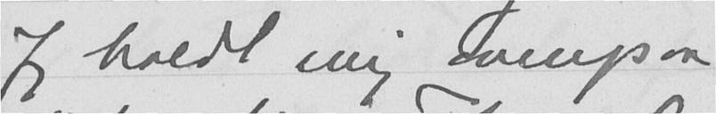Jeg holdt mig ovenpaa
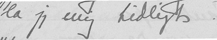la jeg mig tidligt.
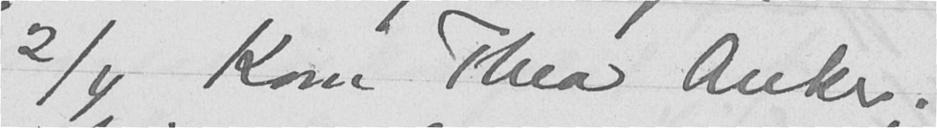2/4 Kom Thea Anker.
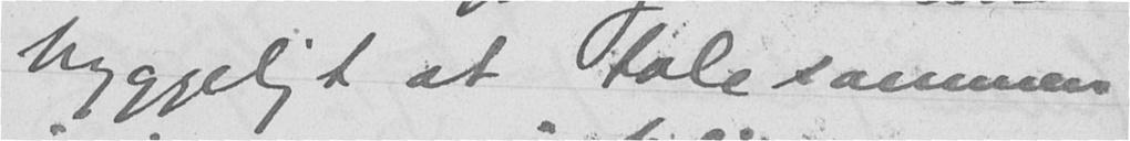hyggeligt at tale sammen
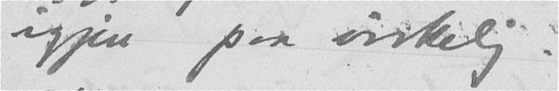igjen paa virkelig.
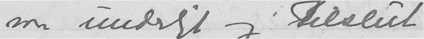var underligt og tilslut
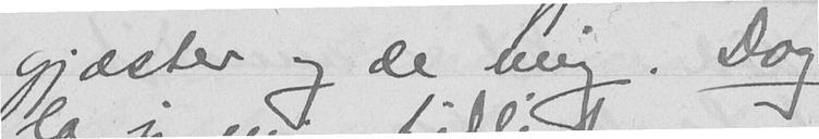gjæster og de mig. Dog
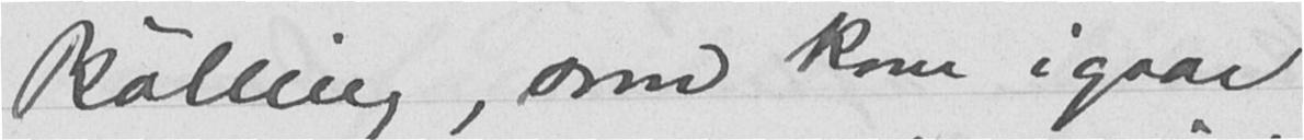Bølling, som kom igaar
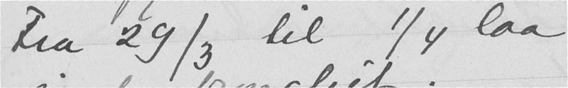Fra 29/3 til 1/4 laa
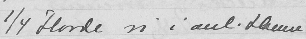1/4 Havde vi i anl. Hanna
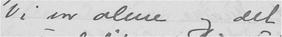Vi var alene og det
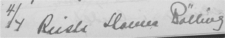4/4 Reiste Hanna Bølling
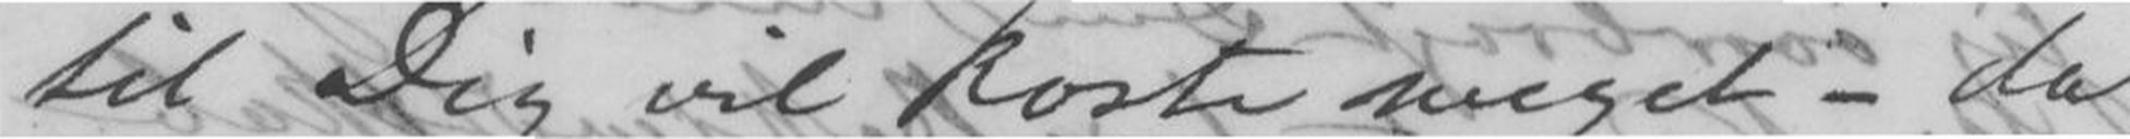til Dig vil koste meget - da
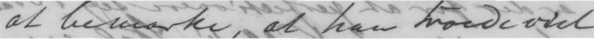at bemærke, at han havde vist
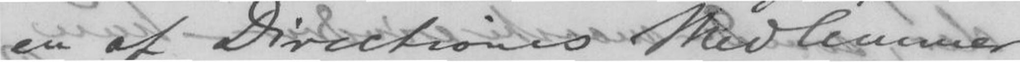en af Directiones Medlemmer
